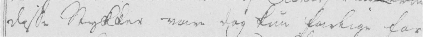disse Stykker vare dog kun farlige for
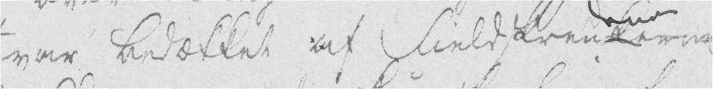var bedækket af Fieldskrenter
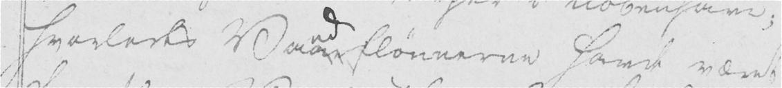hvorledes Vaarfløerne havde været
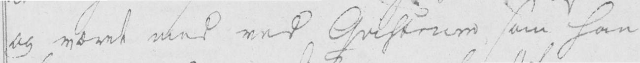og været med ved Qvistrum som han
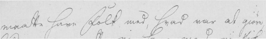maatte have Folk med, hvad var at giøre,
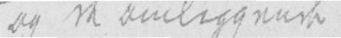og de omliggende
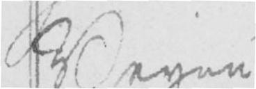Veyen
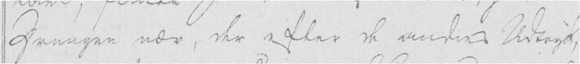Drengen nær, der efter de andres Udtryk,
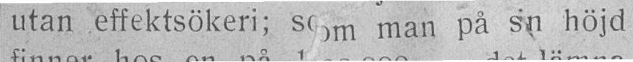utan effektsökeri; som man på sn höjd
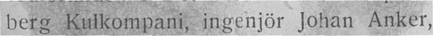berg Kulkompani, ingenjör Johan Anker,
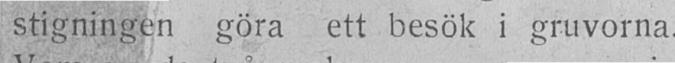stigningen göra ett besök i gruvorna.
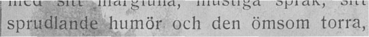sprudlande humör och den ömsom torra,
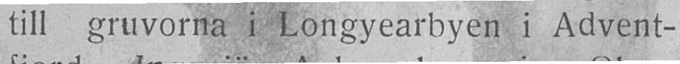till gruvorna i Longyearbyen i Advent-
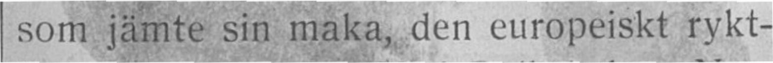som jämte sin maka, den europeiskt rykt-
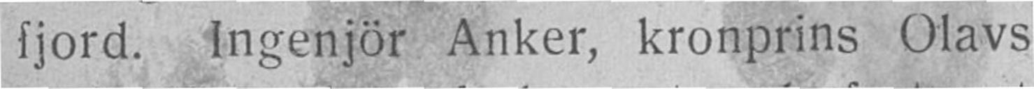fjord. Ingenjör Anker, kronprins Olavs
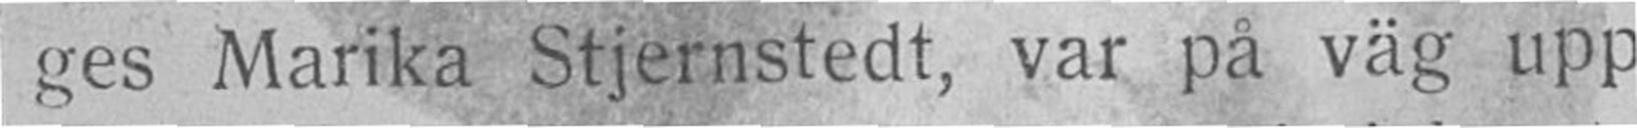ges Marika Stjernstedt, var på väg upp
